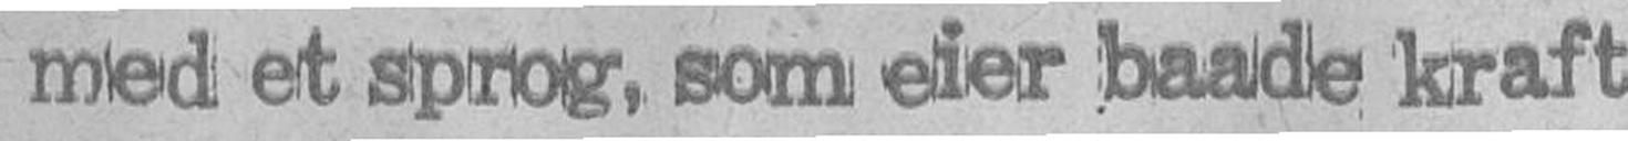med et sprog, som eier baade kraft
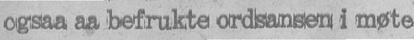ogsaa aa befrukte ordsansen i møte
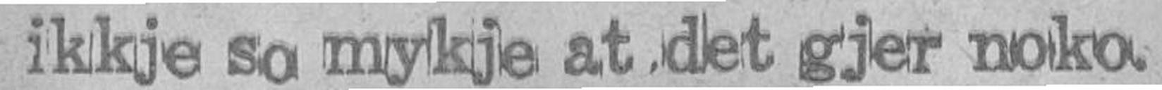ikkje so mykje at det gjer noko.
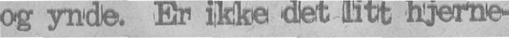og ynde. Er ikke det litt hjerne-
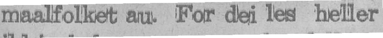maalfolket au. For dei les heller
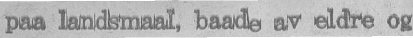paa landsmaal, baade av eldre og
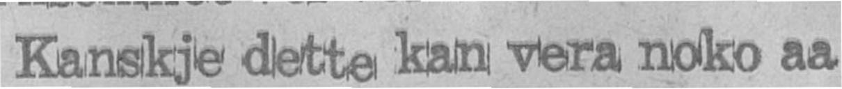Kanskje dette kan vera noko aa
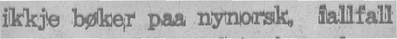ikkje bøker paa nynorsk, tallfall
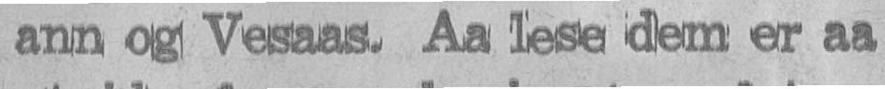ann og Vesaas. Aa lese dem er aa
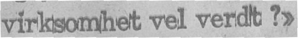virksomhet vel verdt ?»
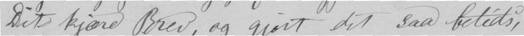Dit kjære Brev, og gjort det saa betids,
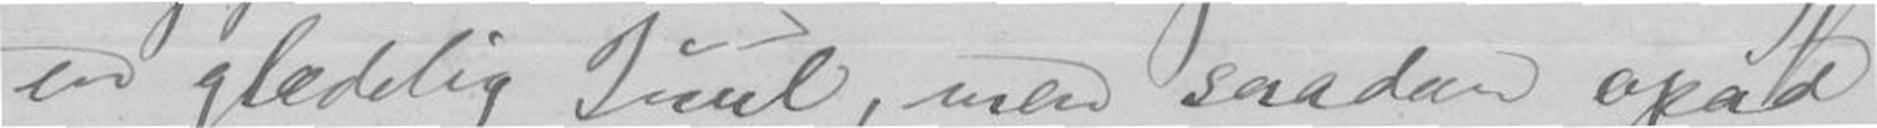en glædelig Juul, men saadan opad
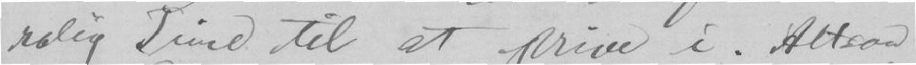rolig Time til at skrive i. Altsaa
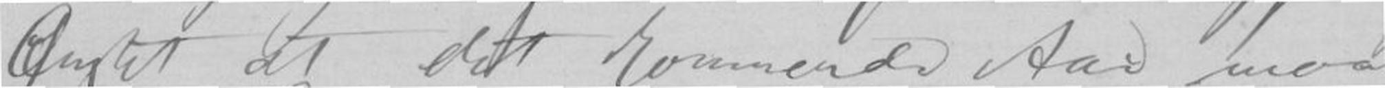Ønsket at det kommende Aar maa
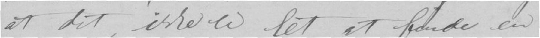at det ikke er let at finde en
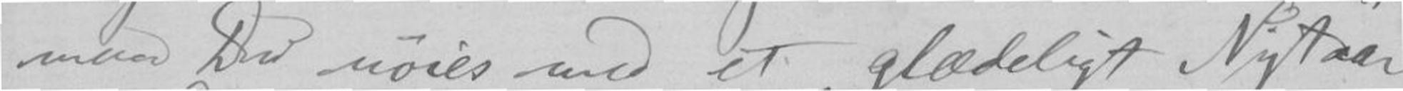maa Du nøies med et glædeligt Nytaar
